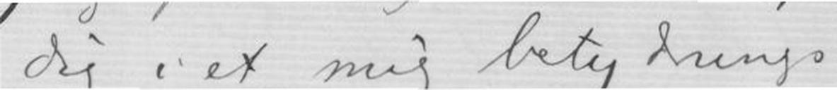dig i et mig betydnings
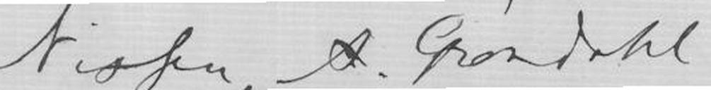Nissen, A Grøndahl
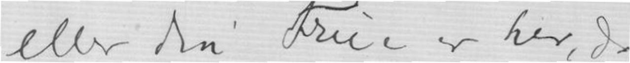eller Din Frue er her, da
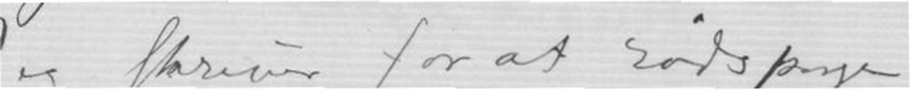Jeg skriver for at rådspørge
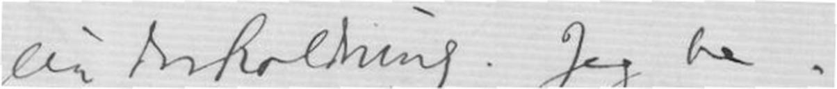underholdning. Jeg be-
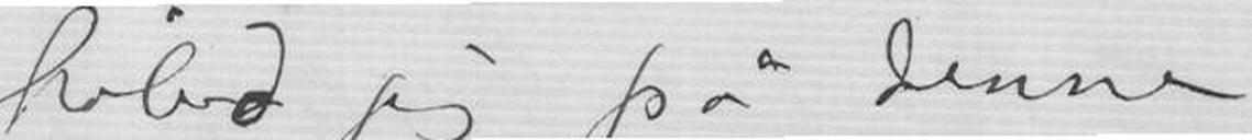håbed jeg på denne
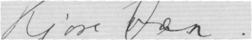Kjære Ven!
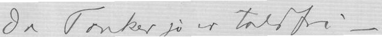da Tanker jo er toldfri
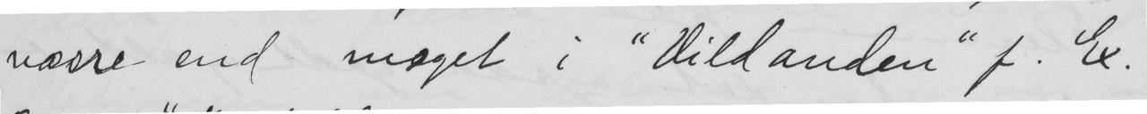værre end moget i "Vildanden" f. Ex.
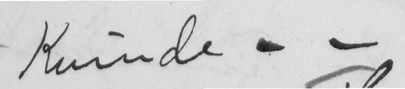Kvinde - -
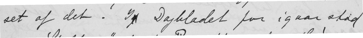set af det. I Dagbladet for igaar stod
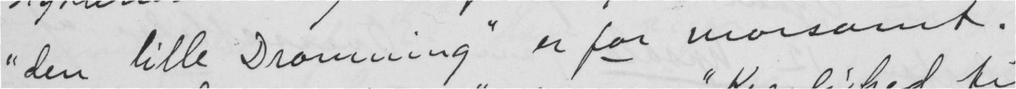"den lille Dronning" er for morsomt.
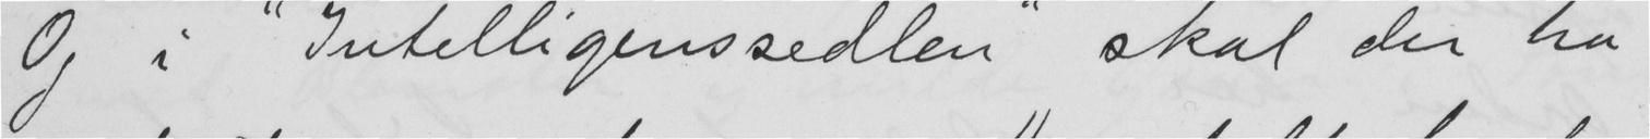Og i "Jutelligenssedlen" skal der ha
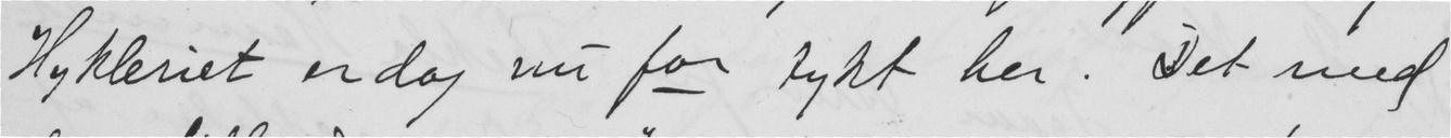Hykleriet er dog nu for tykt her. Det med
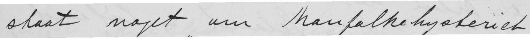staat noget om Manfolkehysteriet
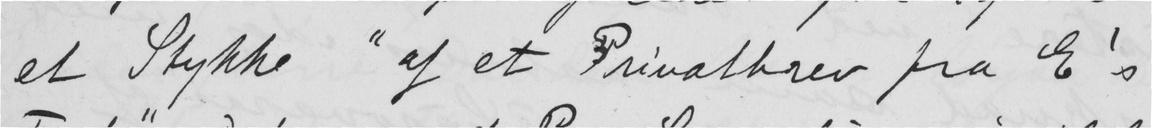et Stykke "af et Privatbrev fra E's
